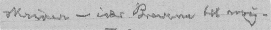skriver - især Brevene til mig.
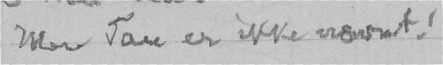Men Tau er ikke nævnt!
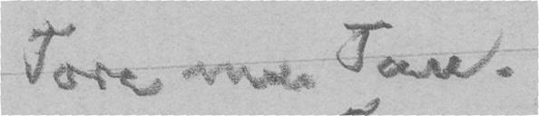Tore men Tau.
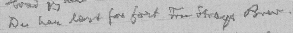Du har læst for fort Fru Strays Brev.
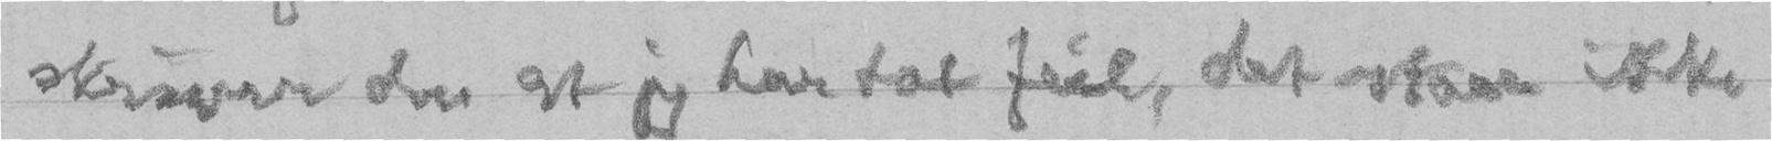skriver du at jeg har tat feil, det staar ikke
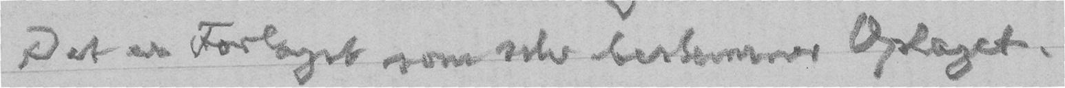Det er Forlaget som selv bestemmer Oplaget.
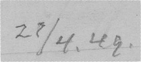29/4. 49.
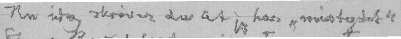Nu idag skriver du at jeg har "mistydet"
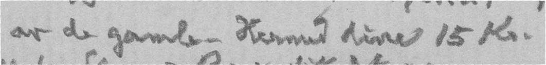av de gamle. Hermed dine 15 Kr.
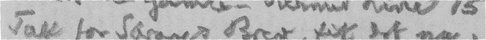Tak for Strays Brev, fik det nu.
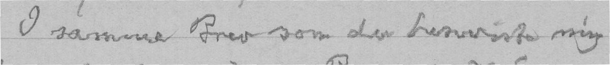I samme Brev som du henviste mig
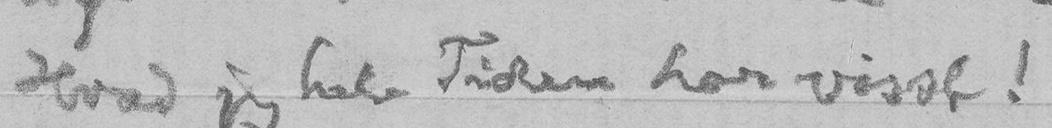Hvad jeg hele Tiden har visst!
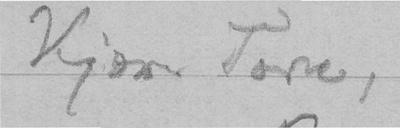Kjære Tore,
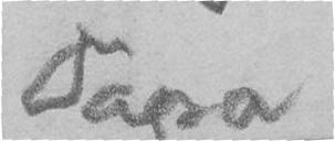Papa
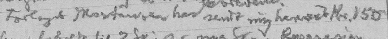Forlaget Mortensen har sendt mig herved Kr. 150
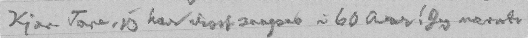Kjære Tore, jeg har visst saapas i 60 Aar! Jeg nævnte
